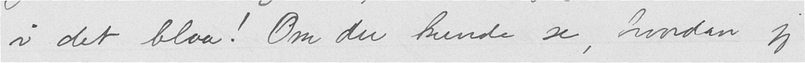i det blaa! Om du kunde se, hvordan jeg
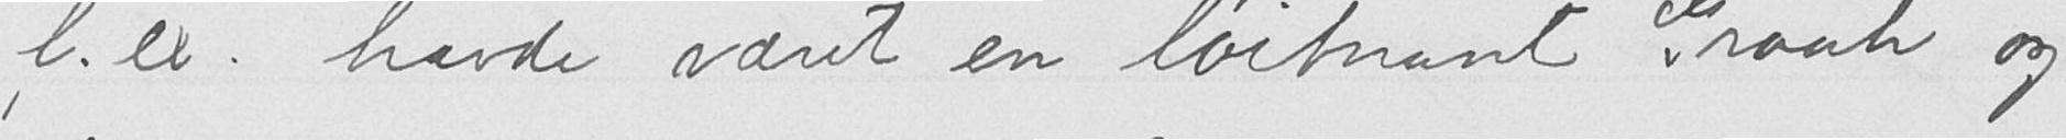f.ex. havde været en løitnant Graah og
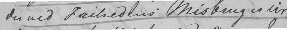der ved Frihedens Misbrug er Vir-
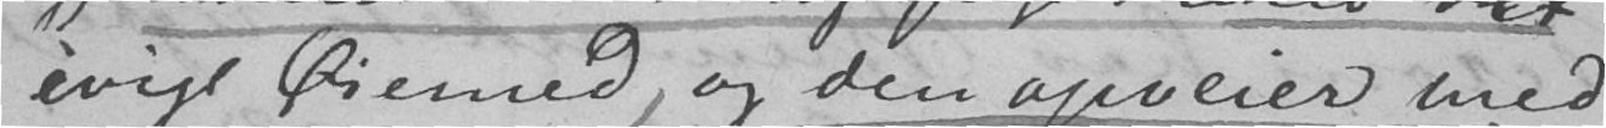evige Øiemed, og den opveier med
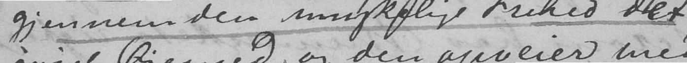gjennem den menneskelige Frihed det
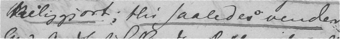keliggjort; thi saaledes vender
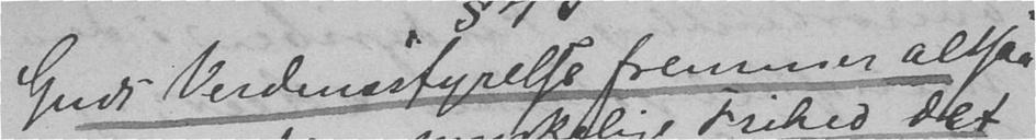Guds Verdensstyrelse fremmer altsaa
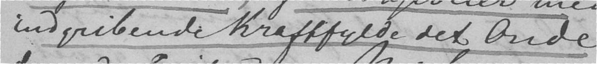indgribende Kraftfylde det Onde
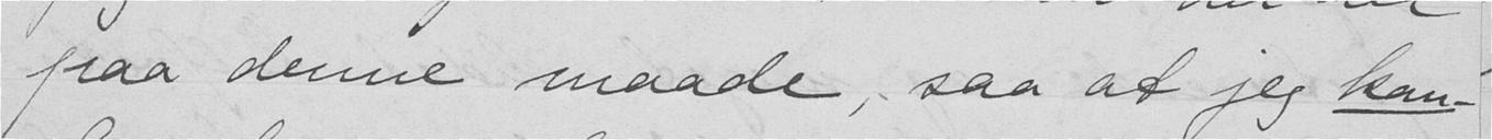paa denne maade, saa at jeg kan-
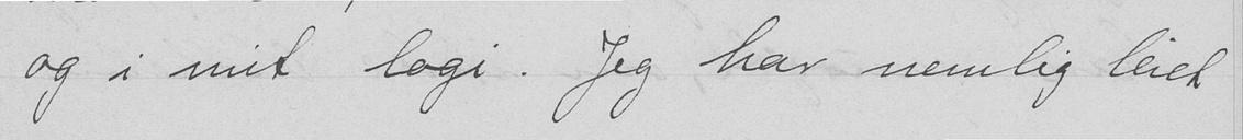og i mit logi. Jeg har nemlig leiet
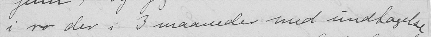i ro der i 3 maaneder med undtagelse
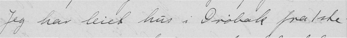Jeg har leiet hus i Drøbak fra 1ste
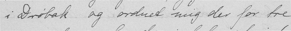i Drøbak, og ordnet mig der for tre
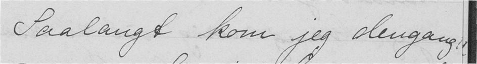Saalangt kom jeg dengang!
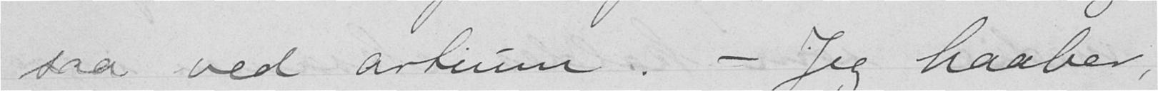saa ved artium. - Jeg haaber,
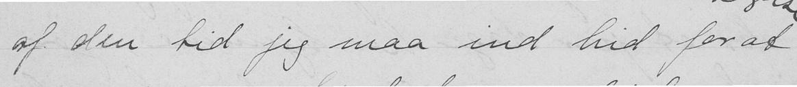af den tid jeg maa ind hid for at
